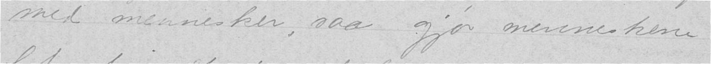med mennesker, saa gjør menneskene
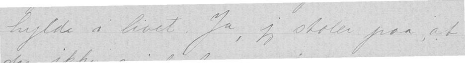hylde i livet. Ja, jeg stoler paa, at
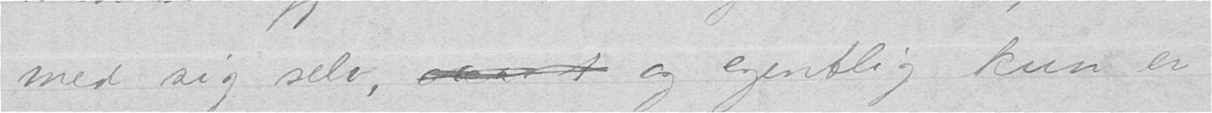med sig selv, og egentlig kun er
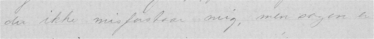du ikke misforstaar mig, men sagen er
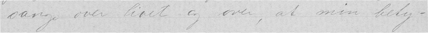sange over livet og over, at min bety-
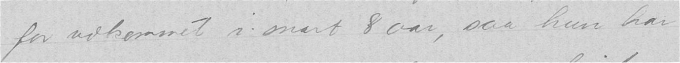for udkommet i snart 8 aar, saa hun har
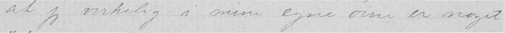at jeg virkelig i mine egne øine er noget
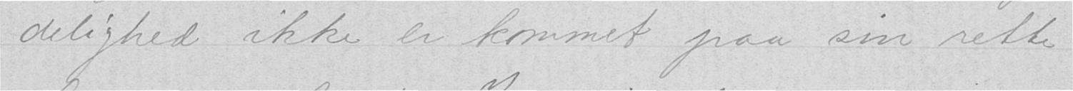delighed ikke er kommet paa sin rette
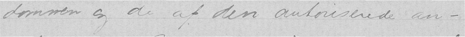dommen og de af den autoriserede an-
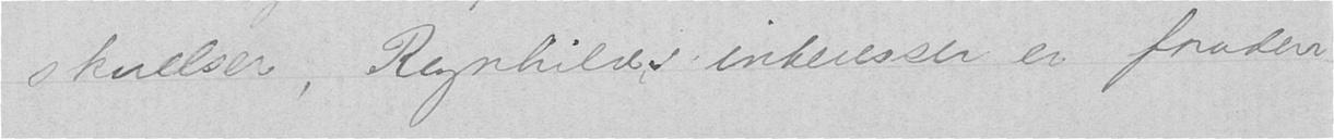skuelser. Ragnhilds interesser er foruden
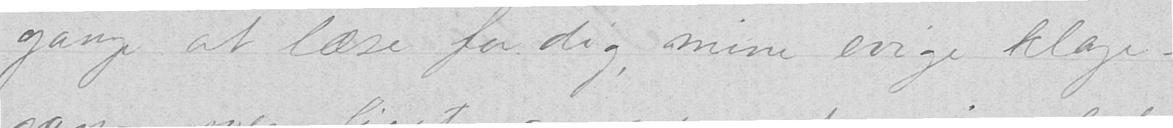gange at læse for dig, mine evige klage-
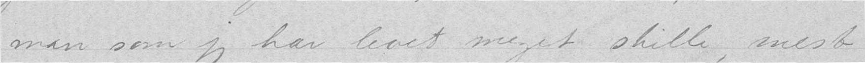man som jeg har levet meget stille, mest
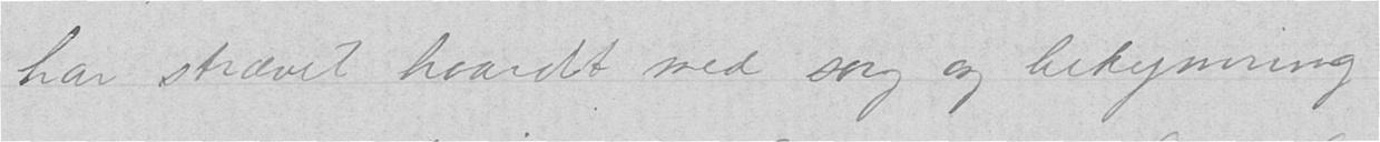har strævet haardt med sorg og bekymring
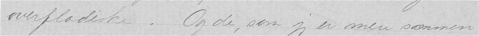overfladiske. Og de, som jeg er mere sammen
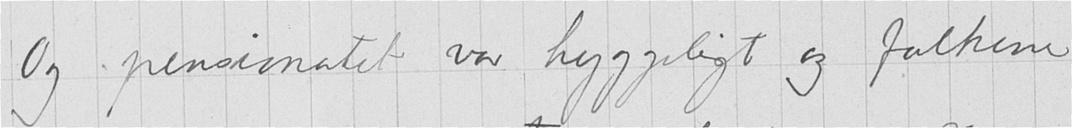Og pensionatet var hyggeligt og folkene
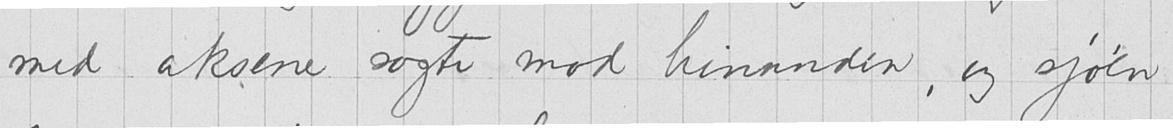med aksene sagte mod hinanden, og sjøen
